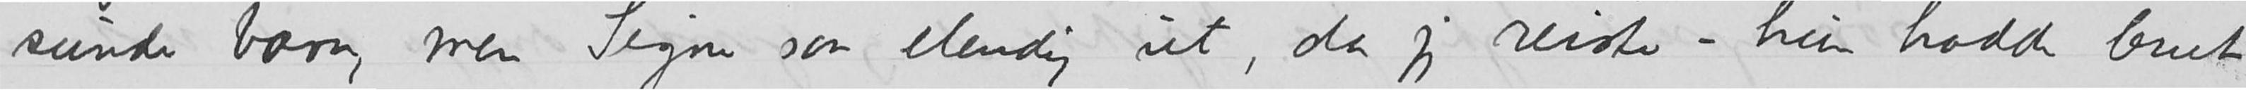sunde barn, men Signe saa elendig ut, da jeg reiste – hun hadde levet
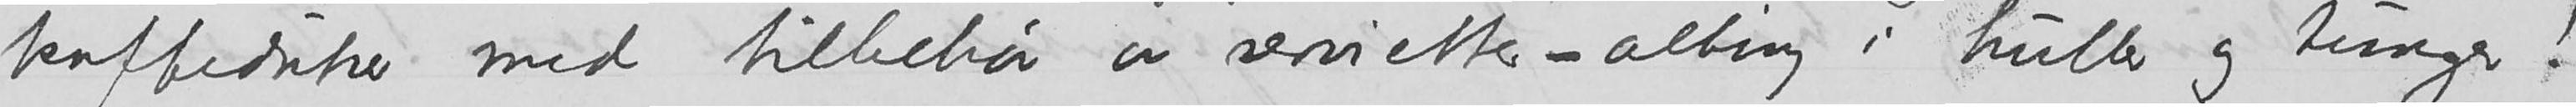kaffeduker med tilbehør av servietter – alting i huller og tunger!
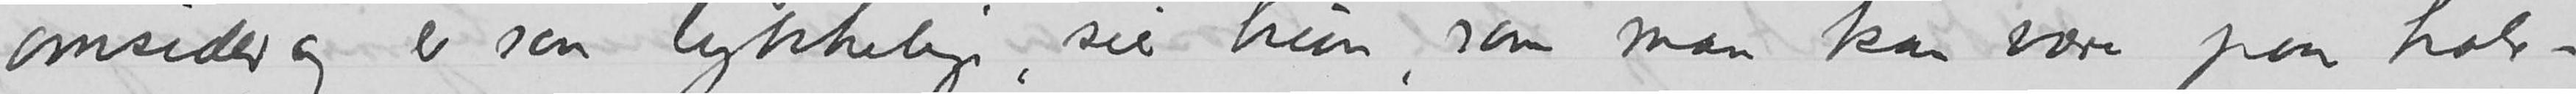omsider og er saa lykkelige, sier hun, som man kan være paa halv-
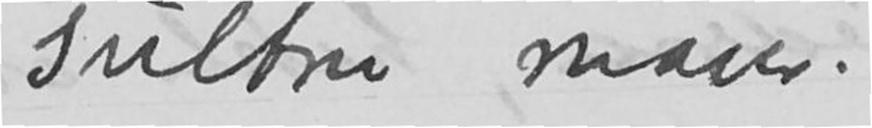sultne maver.
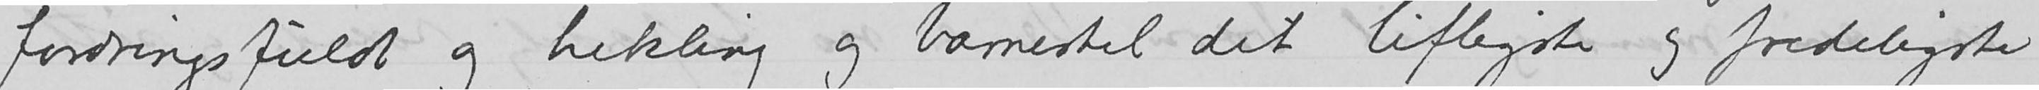fordringsfuldt og hekling og barnestel det lifligste og fredeligste
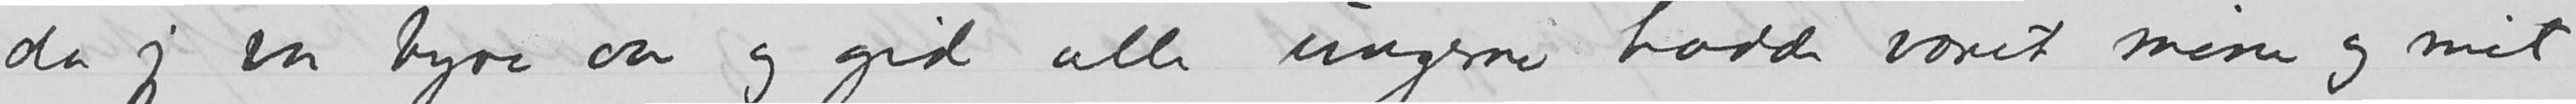da jeg var tyve aar og gid alle ungerne hadde været mine og mit
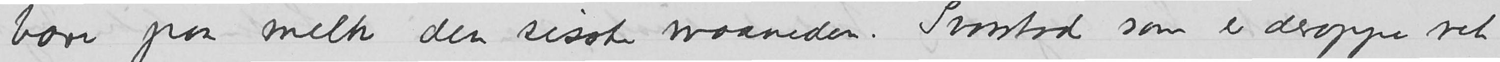bare paa melk den sidste maaneden. Svarstad som er deroppe ret
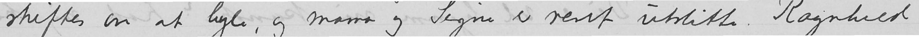skiftes om at hyle, og Mama og Signe er rent utslitte. Ragnhild
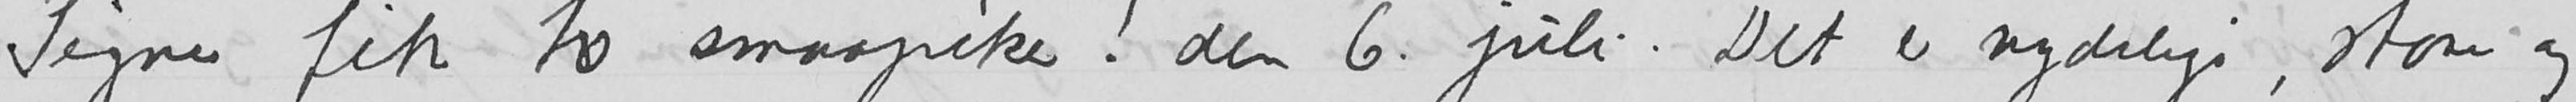Signe fik to smaapiker! den 6. juli. Det er nydelige, store og
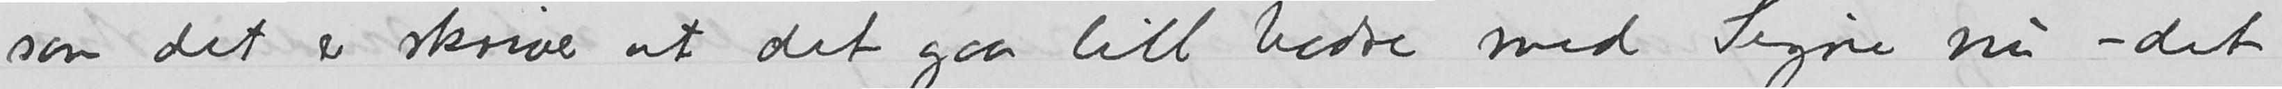som det er skriver at det gaar litt bedre med Signe nu – det
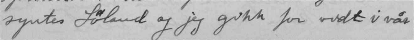syntes Løland og jeg gikk for vidt i vår
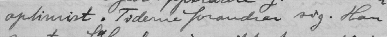optimist. Tiderne forandrer sig. Han
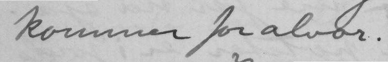kommer for alvor.
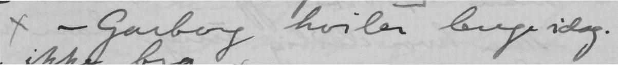x - Garborg hviler lenge idag.
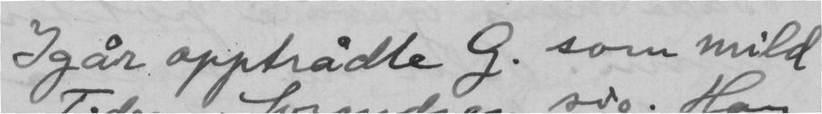Igår opptradte G. som mild
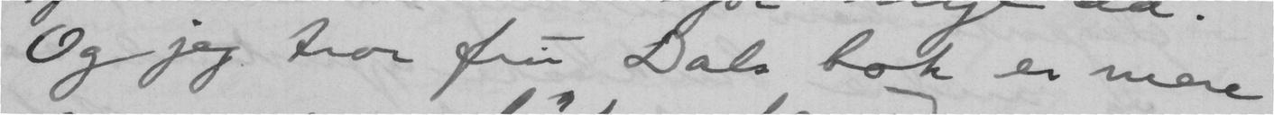Og jeg tror fru Dals bok er mere
